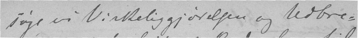søge vi Virkeliggjørelsen og Udbre-
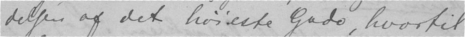delsen af det høieste Gode, hvortil
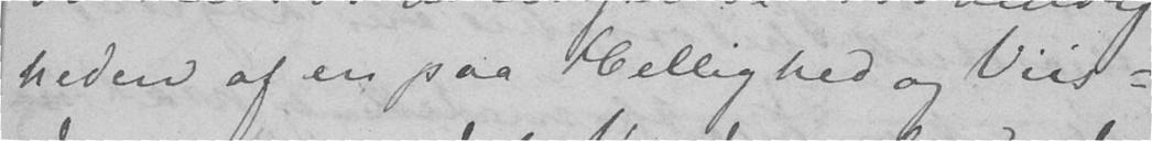heden af en paa Hellighed og Viis-
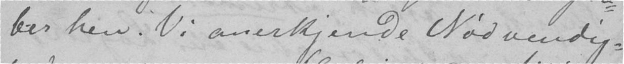ber hen. Vi anerkjende Nødvendig-
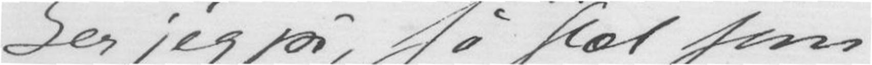ker jeg på, så slæt som
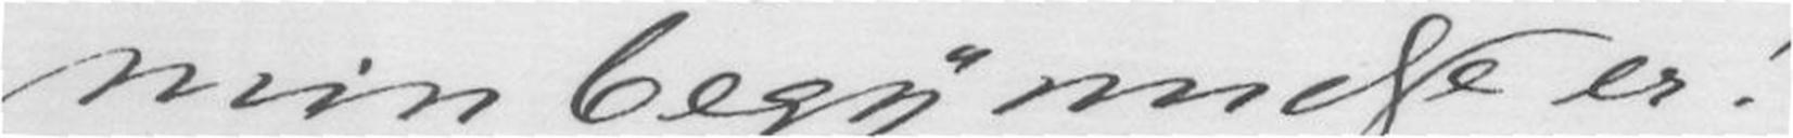min begynnelse er!
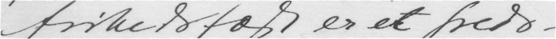frihedsfæst er et freds-
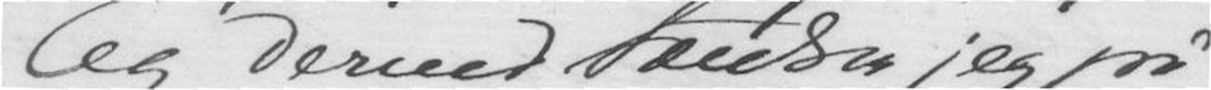Og dermed tænker jeg på
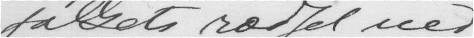folkets rædsel ved
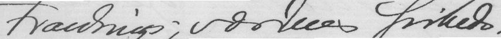Frankrigs, værdens friheds-
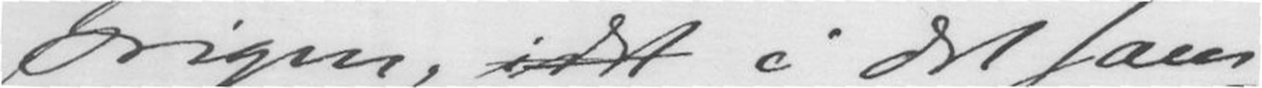krigen, idet i det sam-
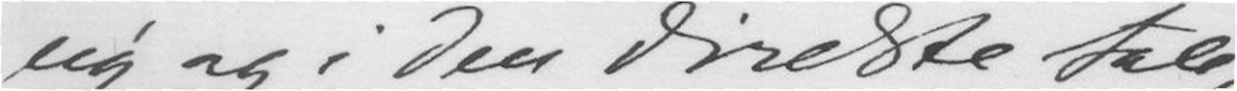ny og i den direkte tale,
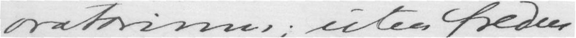oratorium; uten freden
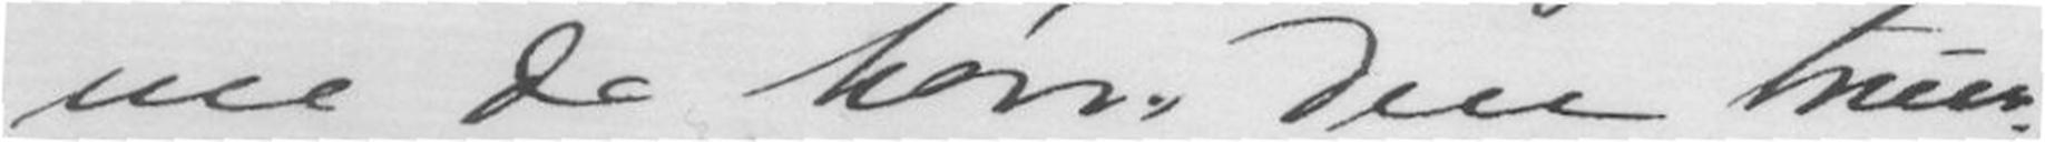me de hører den truer.
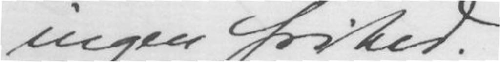ingen frihed.
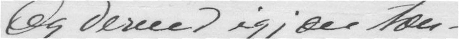Og derved igjæn tæn-
